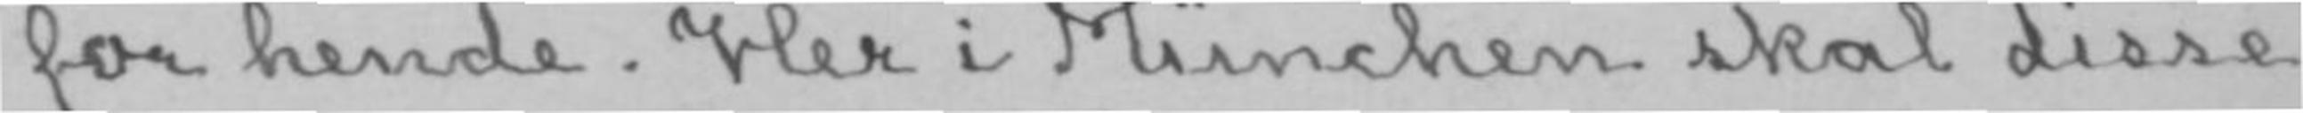for hende. Her i München skal disse
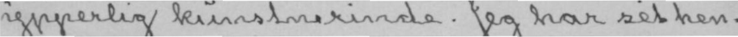ypperlig kunstnerinde. Jeg har sét hen-
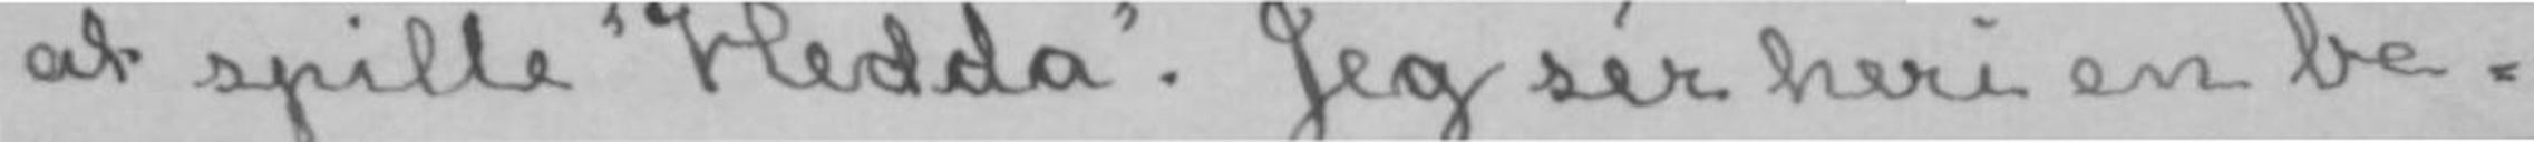at spille «Hedda». Jeg sér heri en be-
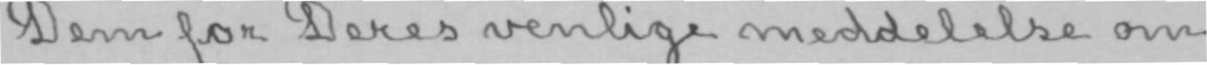Dem for Deres venlige meddelelse om
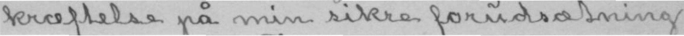kræftelse på min sikre forudsætning
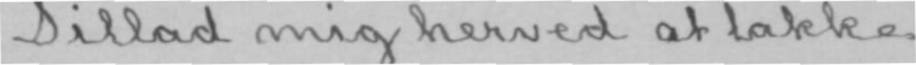Tillad mig herved at takke
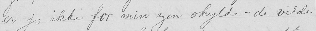er jo ikke for min egen skyld - de vilde
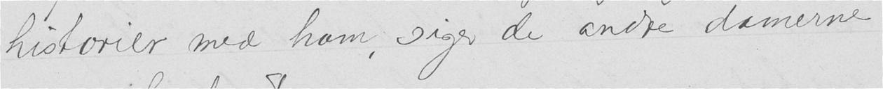historier med ham, siger de andre damerne
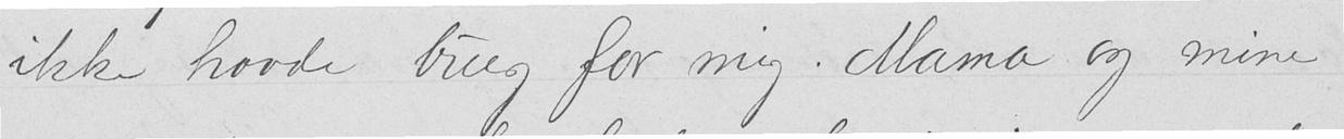ikke havde brug for mig. Mama og mine
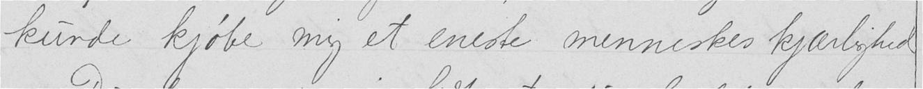kunde kjøbe mig et eneste menneskes kjærlighed.
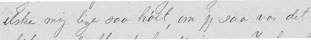elske mig lige saa høit, om jeg saa var det
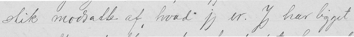stik modsatte af hvad jeg er. Jeg har ligget
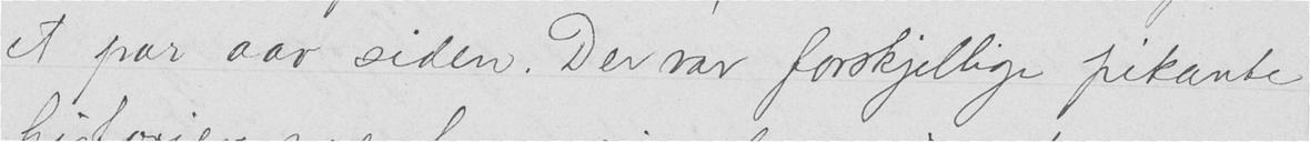et par aar siden. Der var forskjellige pikante
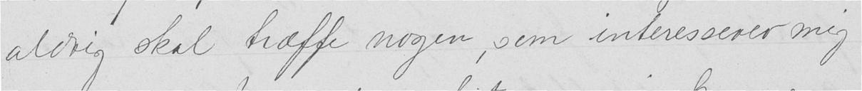aldrig skal træffe nogen, som interesserer mig,
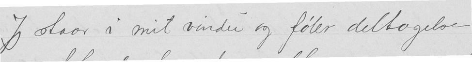Jeg staar i mit vindu og føler deltagelse
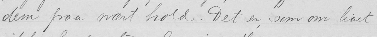dem paa nært hold. Det er, som om livet
